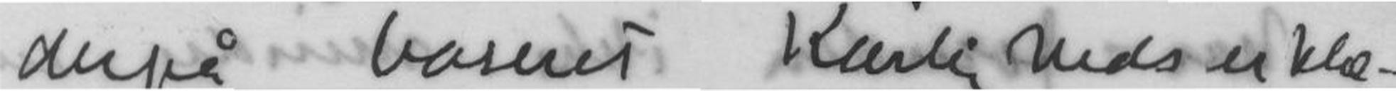derpå baseret Kærligheds erklæ-
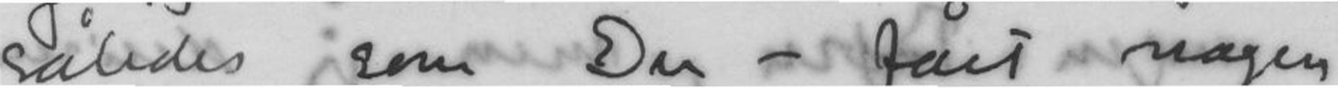således som Du - fået nogen
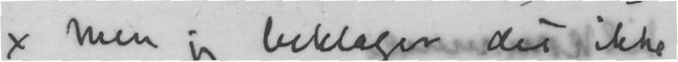x men jeg beklager det ikke
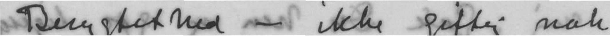Berygtethed - ikke giftig nok
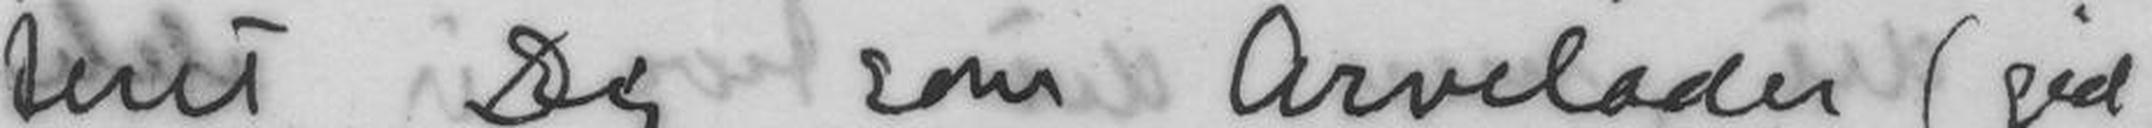teret Dig som Arvelader (gid
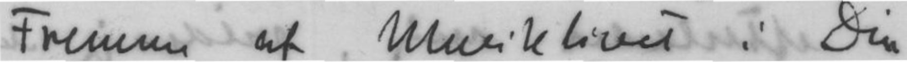Fremme af Musiklivet i Din
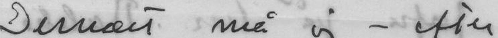Dernæst må jeg - efter
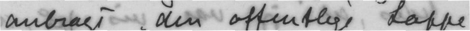anbragt den offentlige Læppe
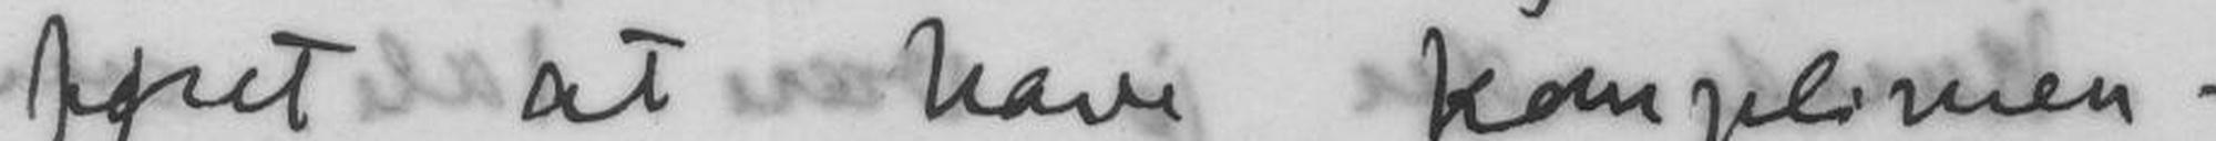først at have komplimen-
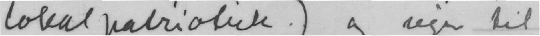lokalpatriotisk) og siger til
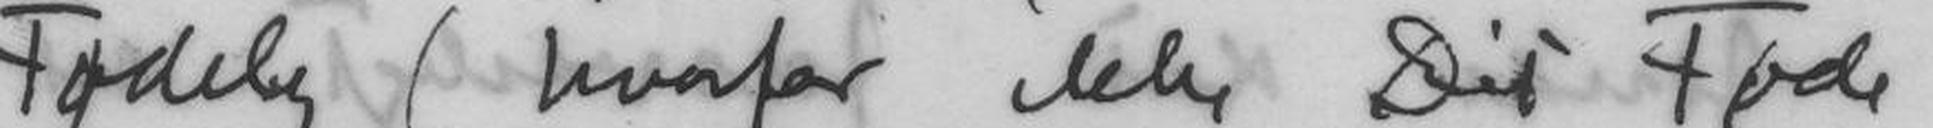Fødeby (hvorfor ikke Dit Føde
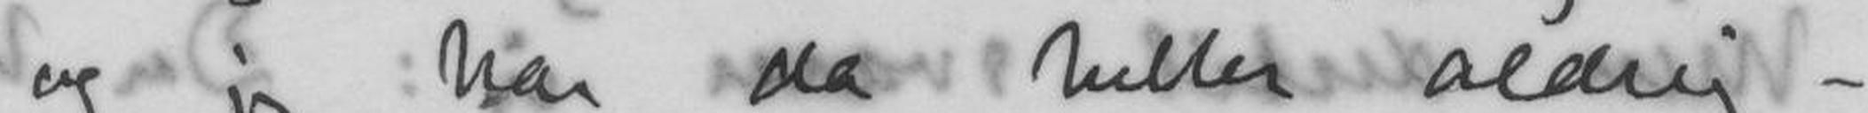og jeg har heller aldrig -
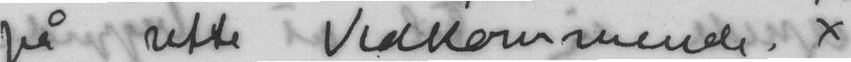på rette Vedkommende. x
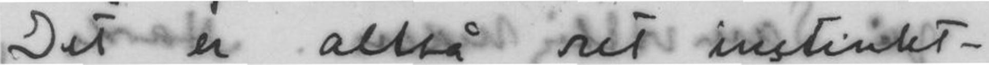Det er altså ret instinkt-
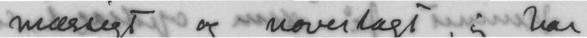mæssigt og uoverlagt, jeg har
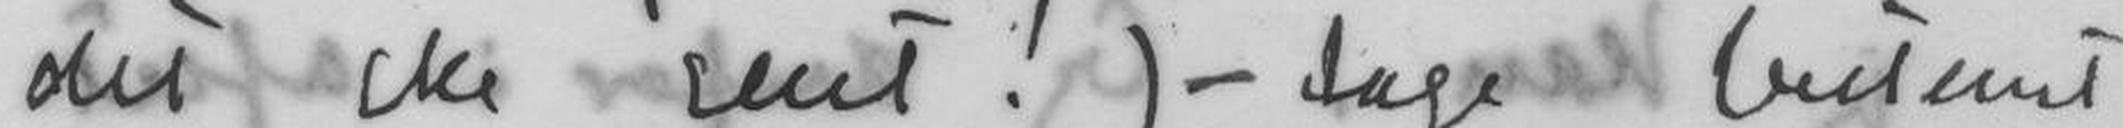det ske sent!) - tage bestemt
